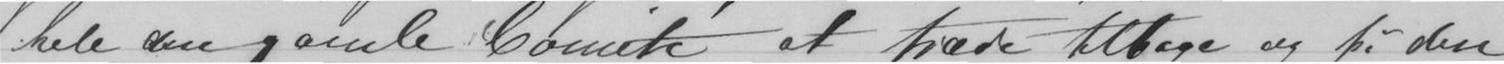hele den gamle Comité at træde tilbage og på den
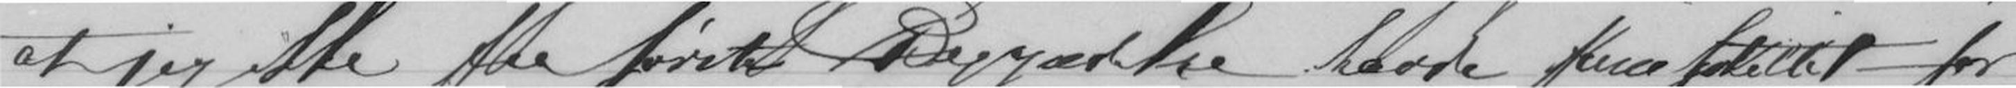at jeg ikke fra første Begyndelse havde fraflittet for
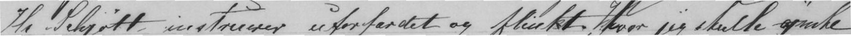Hr Schjøtt instuerer uforfærdet og flinkt, hvor jeg skulde ønske,
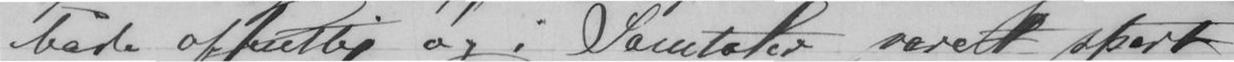både offentlig og i Samtale været spart.
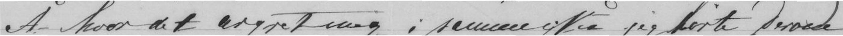Å hvor det ærgret mig i samme Nå jeg hørte dernede,
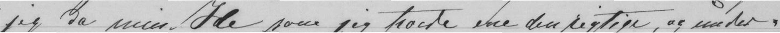jeg da min Ide som jeg troede ene den rigtige, og under-
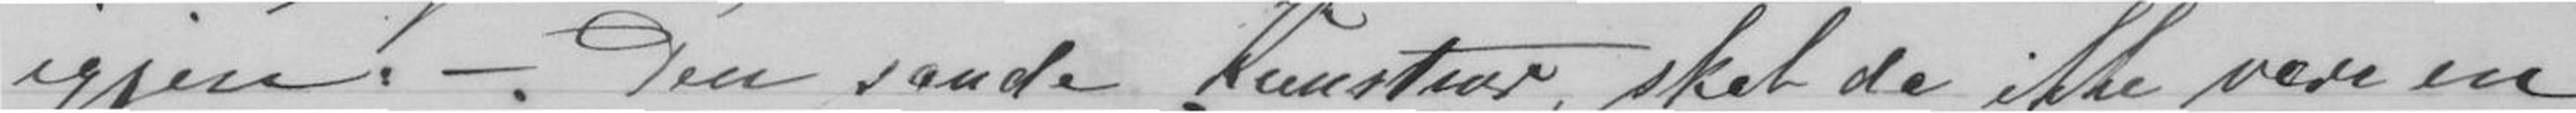igjen. - Den sande Kunstner, skal da ikke være en
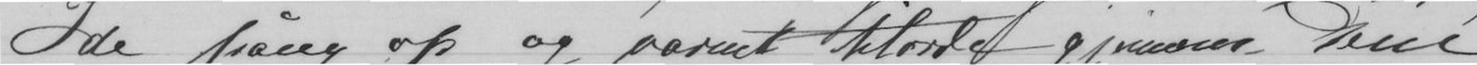Ide påny op og varmt tilorde gjennem Dem,
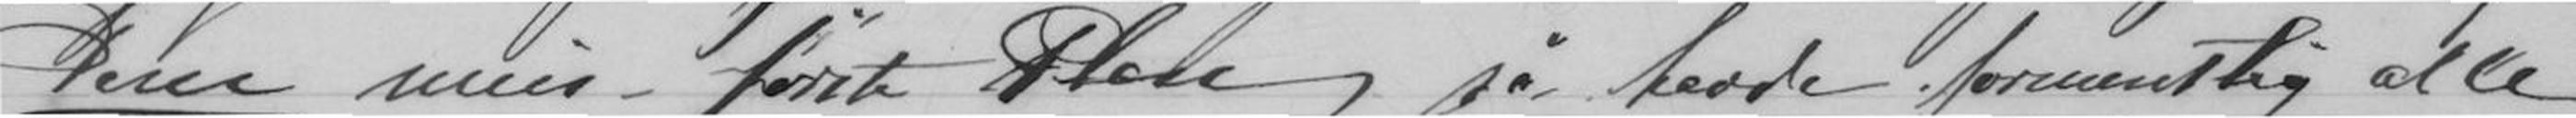Dem min første Plan, så havde formentlig alle
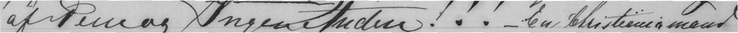af Dem og Ingen Anden!!! - En Christianiamand
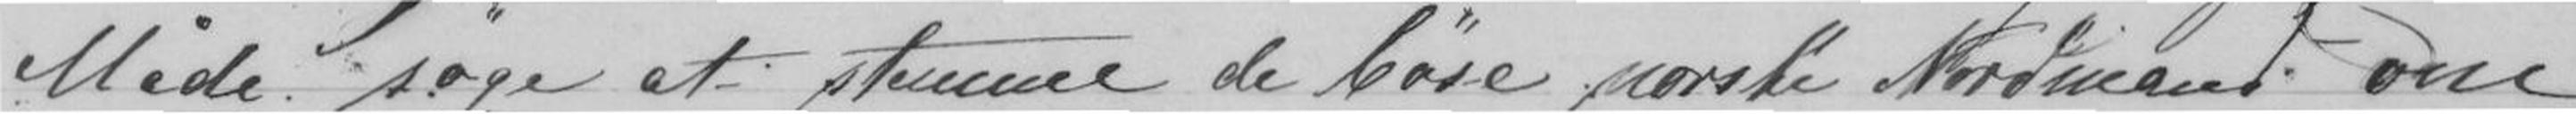Måde søge at stemme de bøse norske Nordmand om
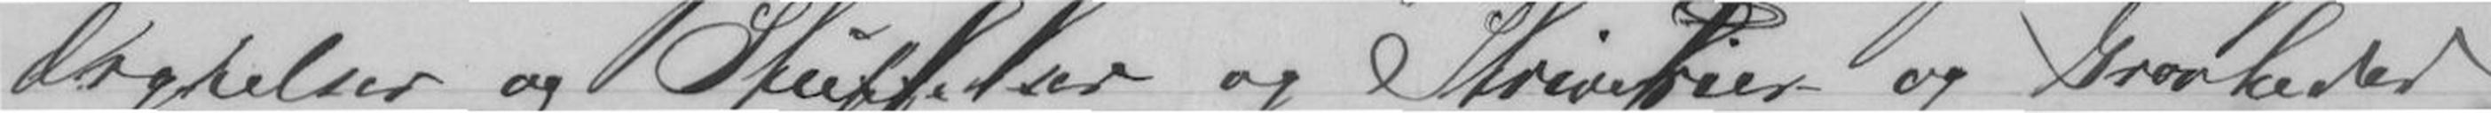Ærgrelser og Skuffelser og Skrivelser og Grovheder
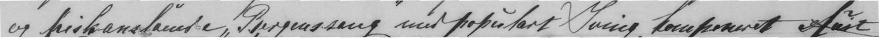og friskanslående "Bergenssang" med populært Sving, komponeret først
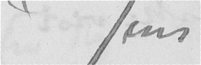Jens
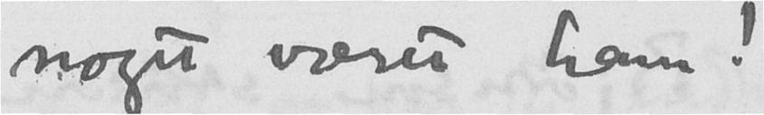noget været ham!
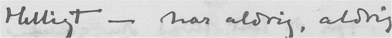Helligt - har aldrig, aldrig
